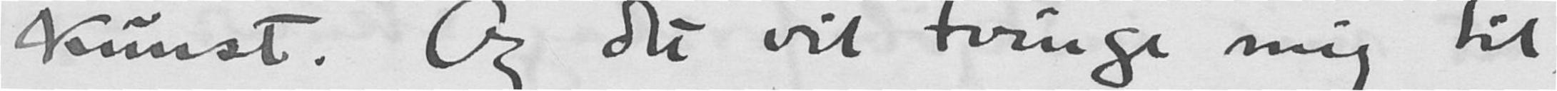kunst. Og det vil tvinge mig til
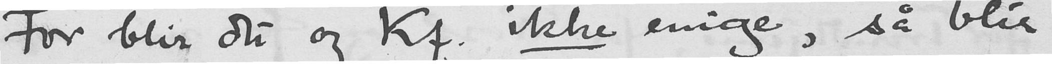For blir du og Kf. ikke enige, så blir
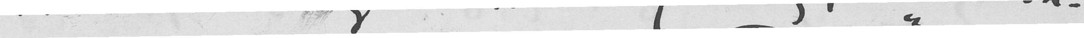medlemskontingent til en forening, hvis virk-
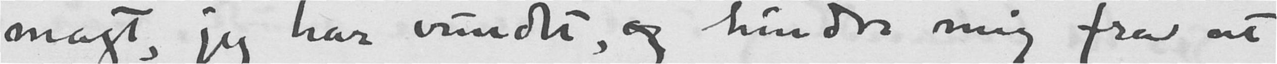magt, jeg har vundet, og hindre mig fra at
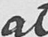at
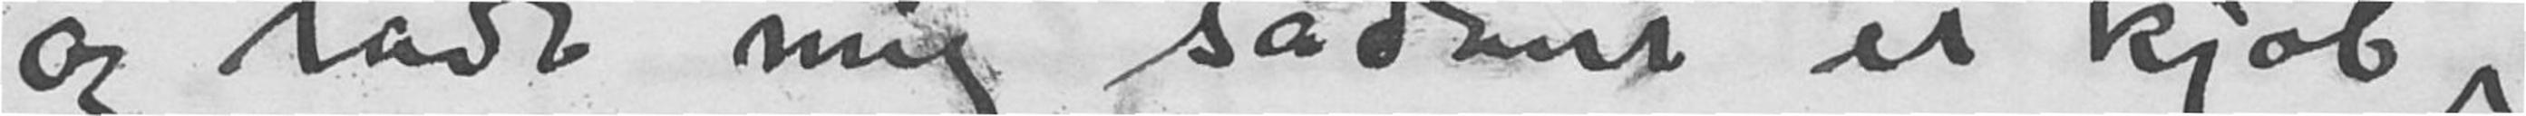og lade mig sådant et kjøb
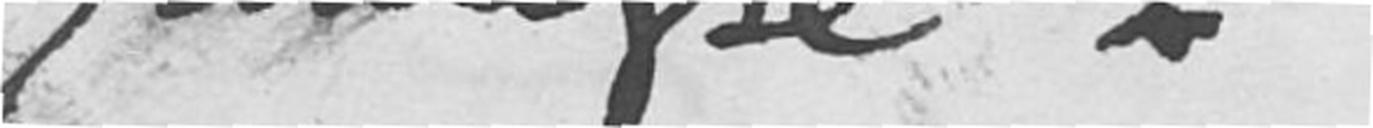rumpe"!
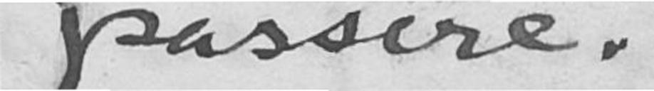passere.
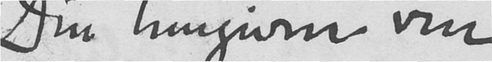Din hengivne ven
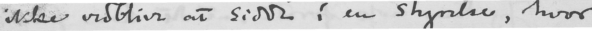ikke vedblive at sidde i en styrelse, hvor
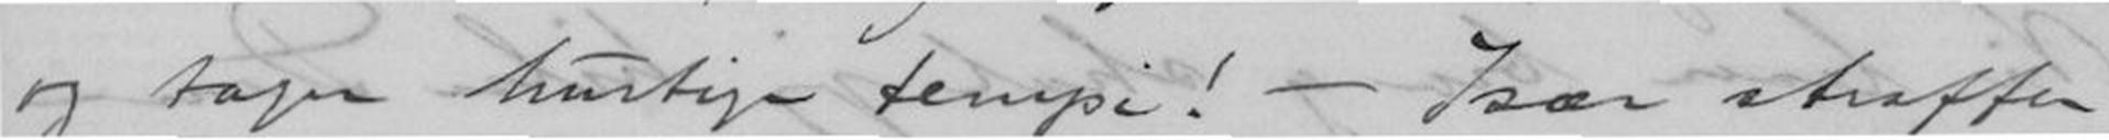og taper hurtige tempi! - Især straffer
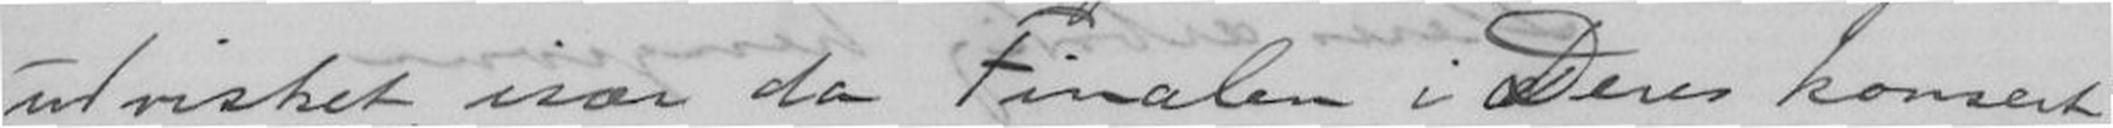udvisket, især da Finalen i Deres konsert
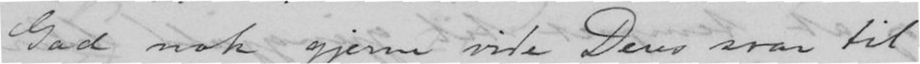Gad nok gjerne vide Deres svar til
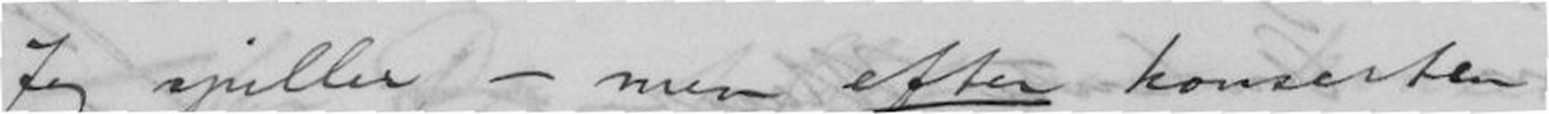Jeg spiller - men efter konserten
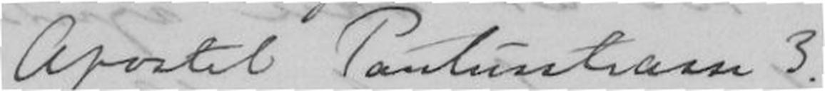Apostel Paulusstrasse 3.
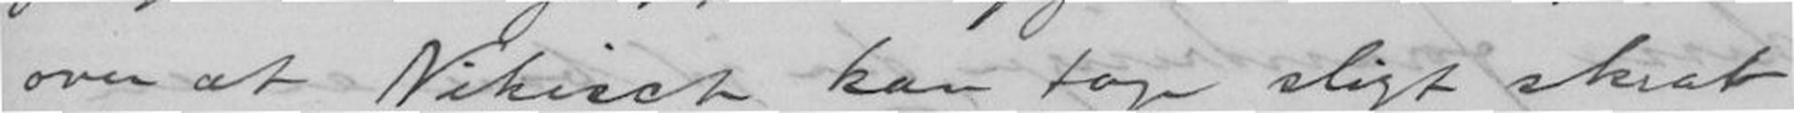over at Nikisch kan tage sligt skrab
